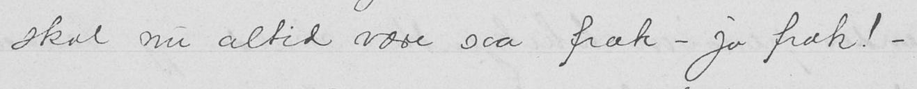skal nu altid være saa fræk — jo fræk! —
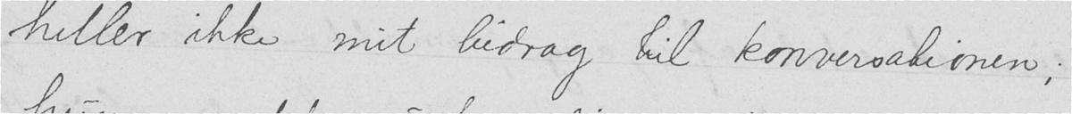heller ikke mit bidrag til konversationen;
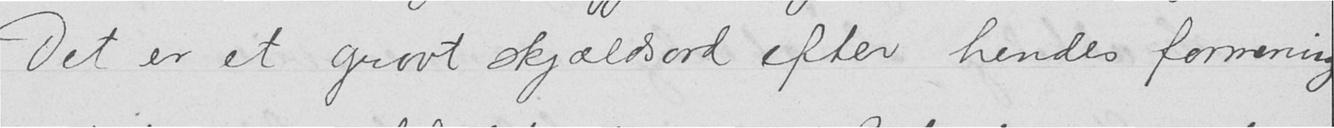Det er et grovt skjældsord efter hendes formening
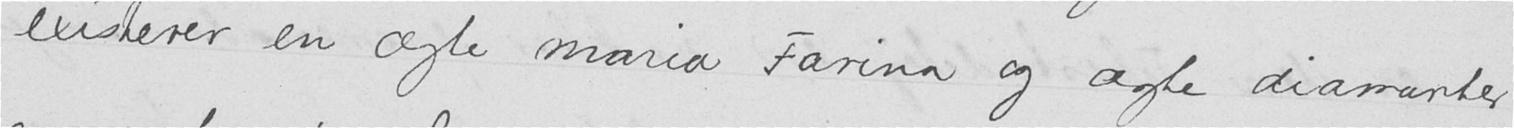existerer en ægte Maria Farina og ægte diamanter
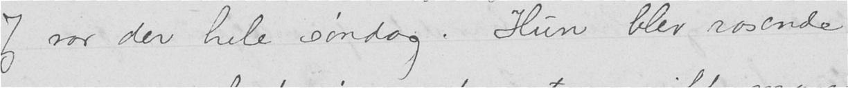Jeg var der hele søndag. Hun blev rasende
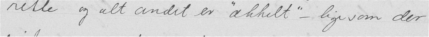rette og alt andet er "ækkelt" — ligesom der
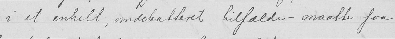— i et enkelt, omdebatteret tilfælde — maatte faa
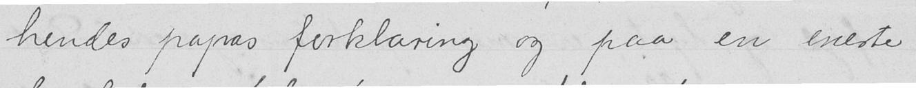hendes papas forklaring og paa en eneste
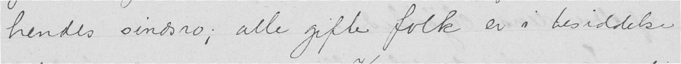hendes sindsro; alle gifte folk er i besiddelse
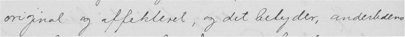original og affekteret, og det betyder, anderledens
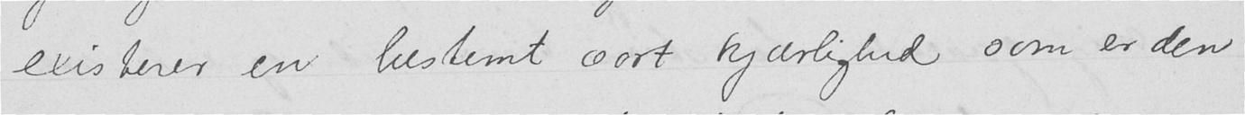existerer en bestemt sort kjærlighed som er den
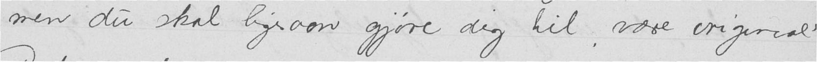men du skal ligesom gjøre dig til, være original"
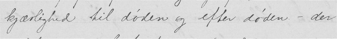kjærlighed til døden og efter døden — der
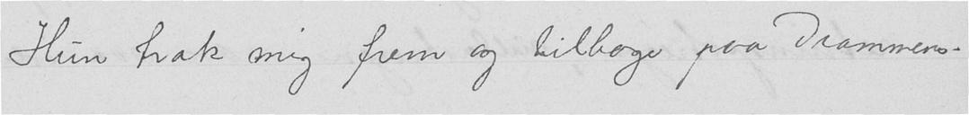Hun trak mig frem og tilbage paa Drammens-
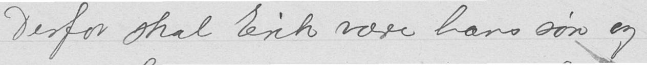Derfor skal Erik være hans søn og
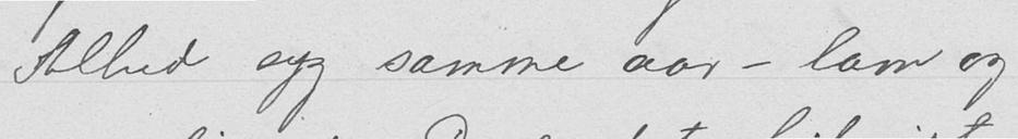Alhed syg samme aar – lam og
